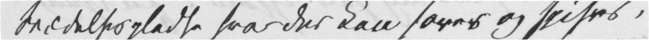trædelsespladse hvor der kan soves og spises,
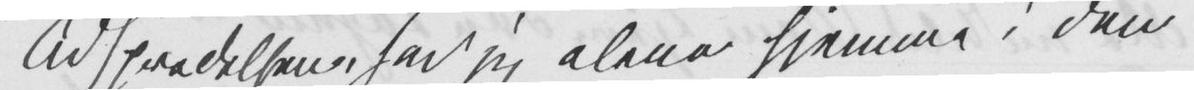Adspredelsene, sad jeg alene hjemme i den
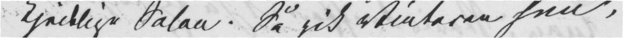kjedelige Salon. Så gik Vinteren hen,
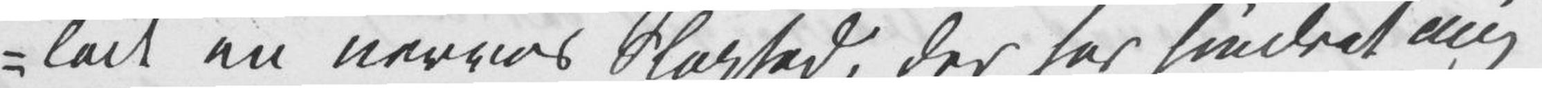ladt en nervøs Slaphed, der har hindret mig
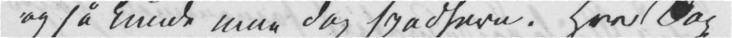og så kunde man dog spadsere. Hver Dag
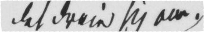det dreier sig om.
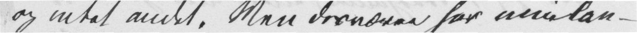og intet andet. Men desværre har min lan=
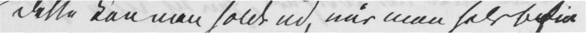Dette kan man holde ud, når man selv befin¬
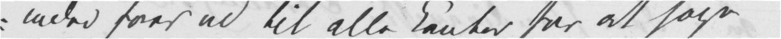inder foer ud til alle Kanter for at søge
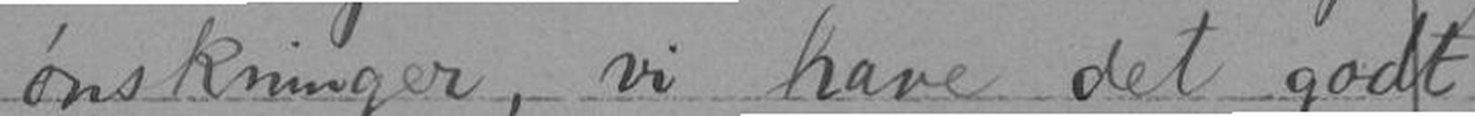ønskninger, vi have det godt,
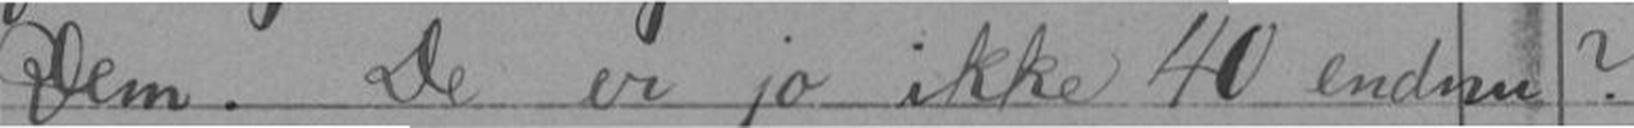Dem. De er jo ikke 40 endnu?
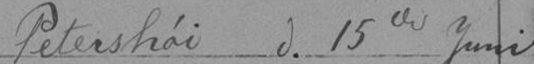Petershøi d. 15de Juni
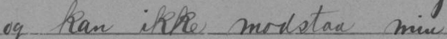og kan ikke modstaa min
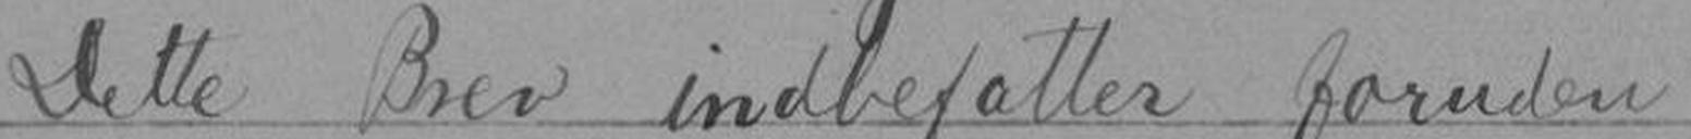Dette Brev indbefatter foruden
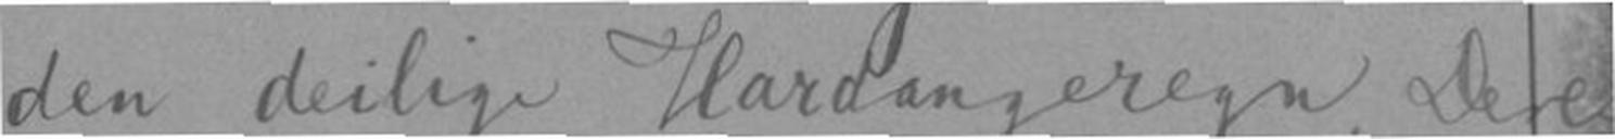den deilige Hardangeregn, Deres
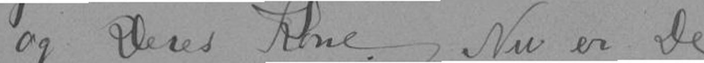og Deres Kone. Nu er De
